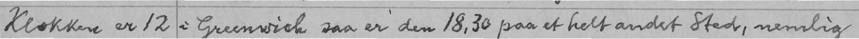Klokken er 12 i Greenwich saa er den 18,30 paa et helt andet Sted, nemlig
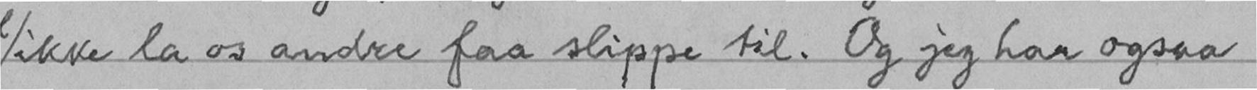ikke la os andre faa slippe til. Og jeg har ogsaa
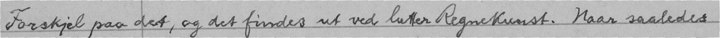Forskjel paa det, og det findes ut ved lutter Regnekunst. Naar saaledes
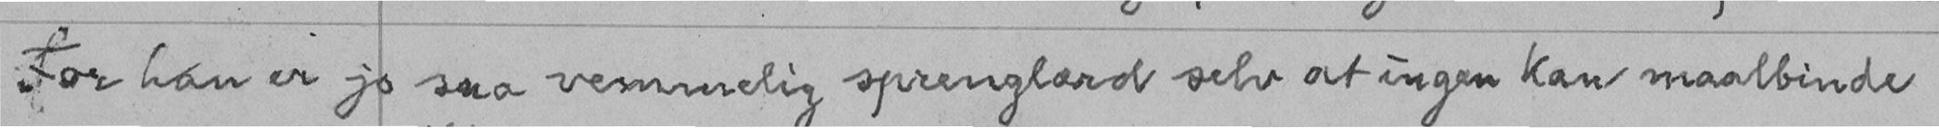For han er jo saa vemmelig sprenglærd selv at ingen kan maalbinde
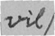vil
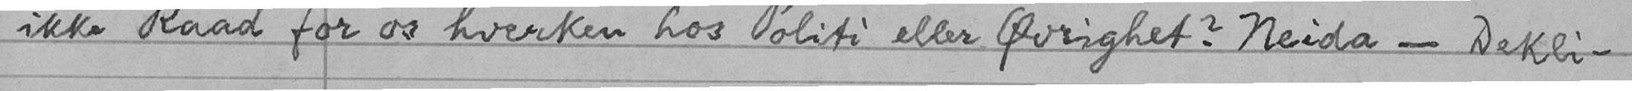ikke Raad for os hverken hos Politi eller Øvrighet? Neida - Dekli-
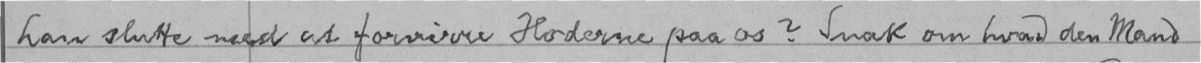han slutte med at forvirre Hoderne paa os? Snak om hvad den Mand
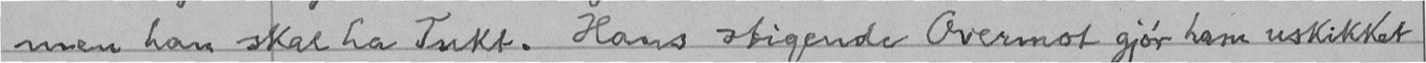men han skal ha Tukt. Hans stigende Overmot gjør ham uskikket
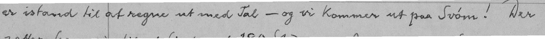er istand til at regne ut med Tal - og vi kommer ut paa Svøm! Der
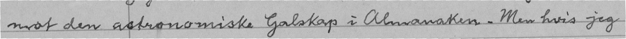mot den astronomiske Galskap i Almanaken. Men hvis jeg
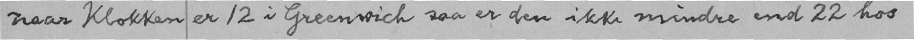naar Klokken er 12 i Greenwich saa er den ikke mindre end 22 hos
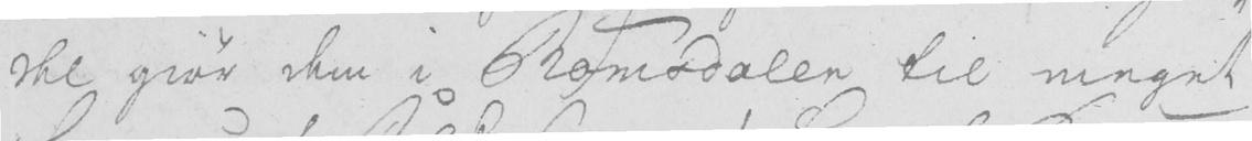del giør dem i Romsdalen til meget
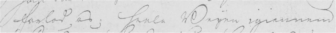forlod os; heele Veyen igiennem
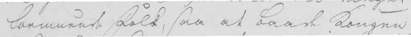formuende Folk, saa at baade Kongen
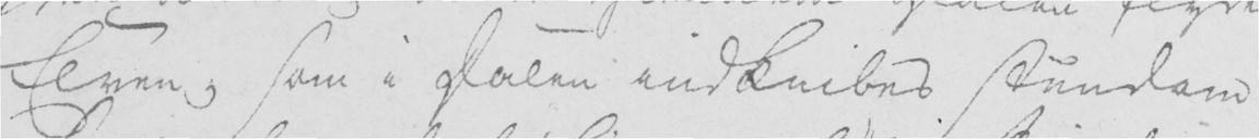Elven, som i Dalen indknibes stundom
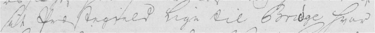sit Præstegield lige til Brøge hvor
